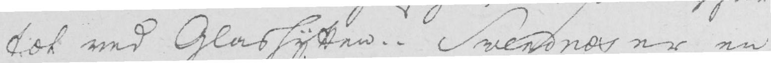tæt ved Glashytten. - Svendnæs er en
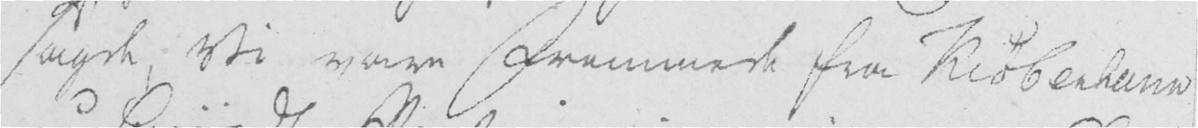sagde, Vi vare Fremmede fra Kiøbenhavn
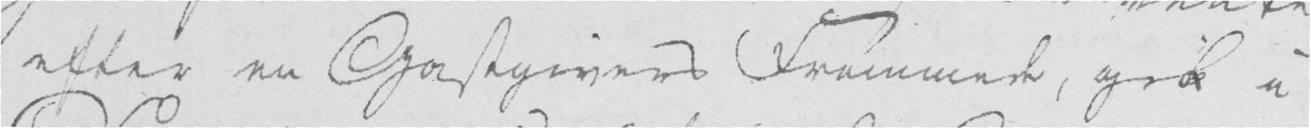efter en Gastgivers Fremmede, gik i
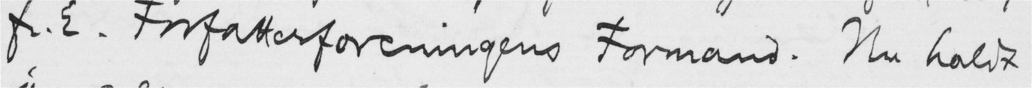f.E. Forfatterforeningens Formand. Nu holdt
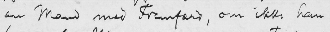en Mand med Fremfærd, om ikke han
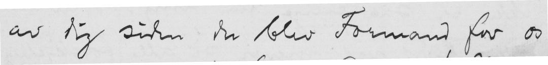av dig siden du blev Formand for os
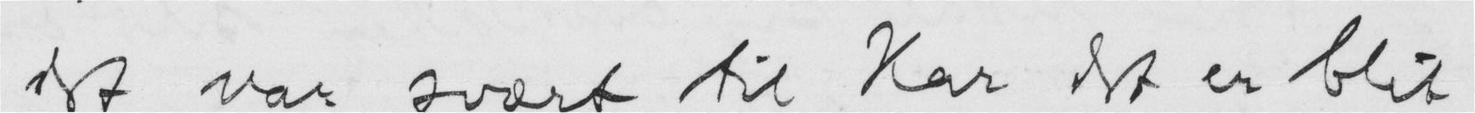det var svært til Kar det er blit
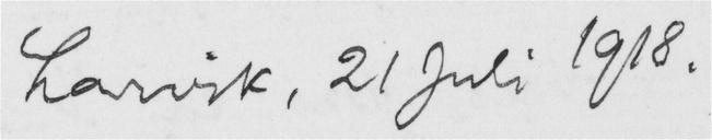Larvik, 21 Juli 1918.
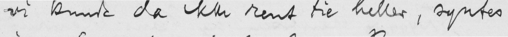vi kunde da ikke rent tie heller, syntes
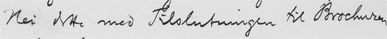Nei dette med Tilslutningen til Brochuren
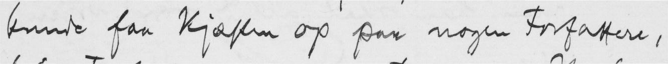kunde faa Kjæften op paa nogen Forfattere,
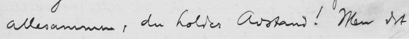allesammen, du holder Avstand! Men det
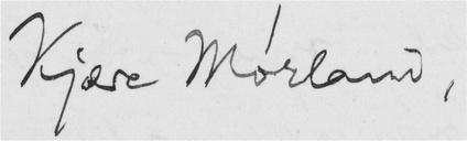Kjære Mørland,
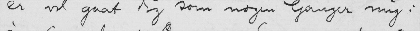er vel gaat dig som nogen Ganger mig:
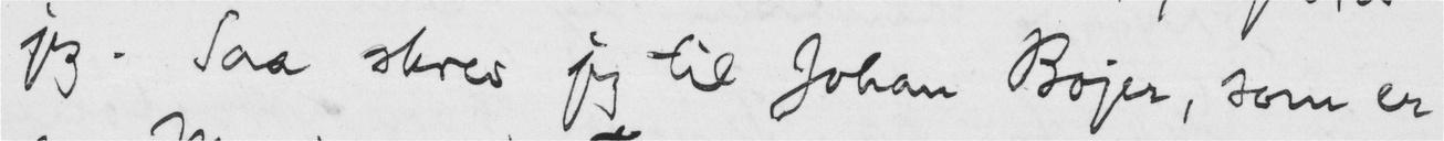jeg. Saa skrev jeg til Johan Bojer, som er
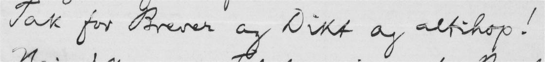Tak for Brever og Dikt og altihop!
